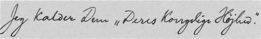Jeg kalder Dem "Deres Kongelige Højhed."
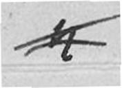#
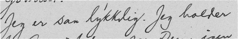Jeg er saa lykkelig. Jeg holder
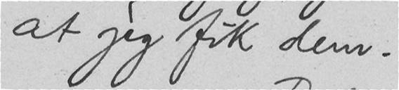at jeg fik dem.
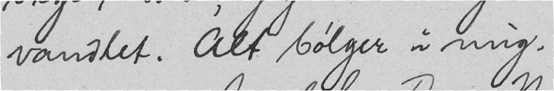vandlet. Alt bølger i mig.
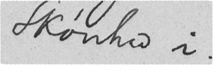Skønhed i.
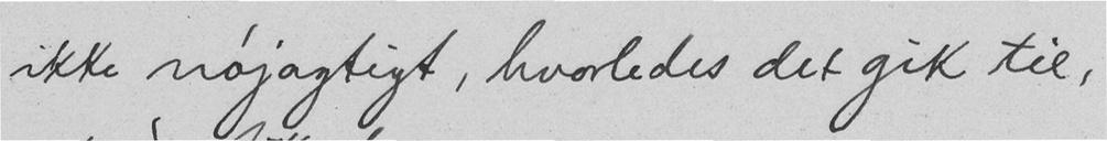ikke nøjagtigt, hvorledes det gik til,
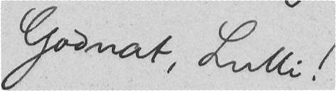Godnat, Lulli!
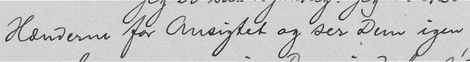Hænderne for Ansigtet og ser Dem igen
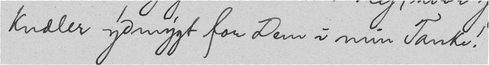knæler ydmygt for Dem i min Tanke!
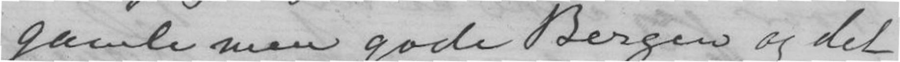gamle men gode Bergen og det
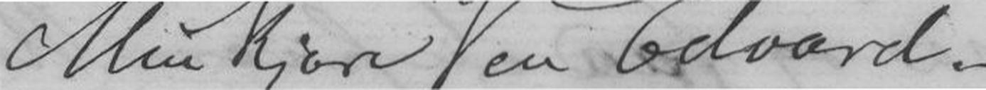Min kjære Ven Edvard.
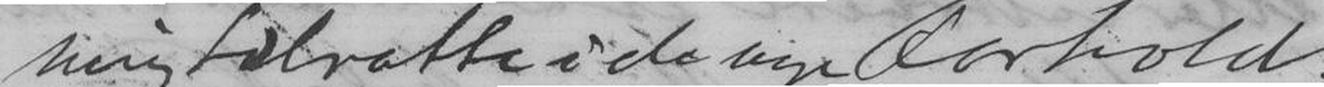mig tilrætte i de nye Forhold.
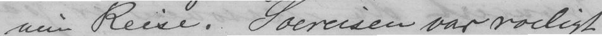min Reise. Soereisen var roeligt
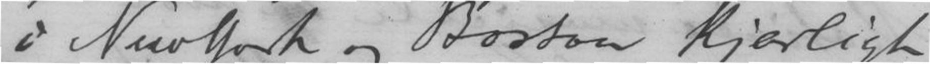i New York og Boston kjærligt
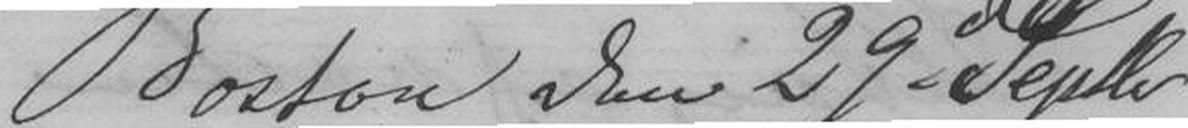Boston den 29d Septbr
